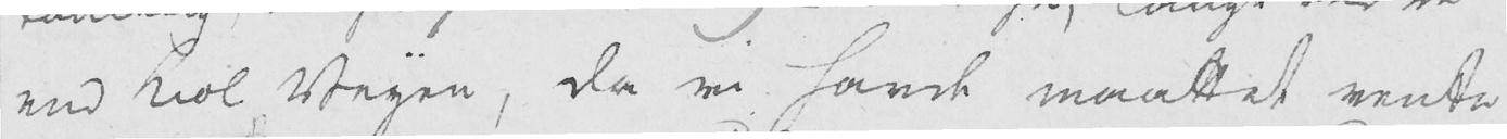end kiøl Veyen, da vi havde maattet vente
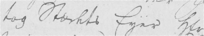tog Stædets Eyer Hr
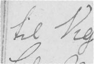til Vig
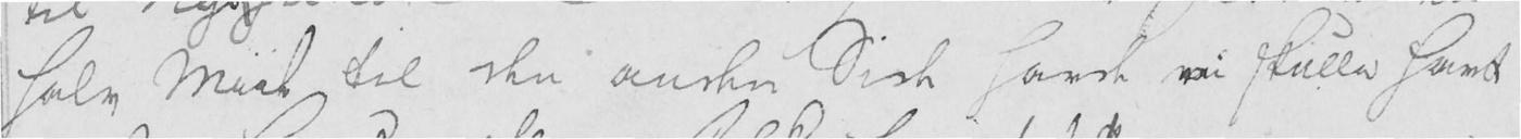halv Miil til den anden Side havde vi skulle havt
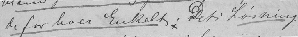de for hver Enkelt. Dets Løsning
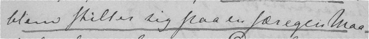blem stiller sig paa en særegen Maa-
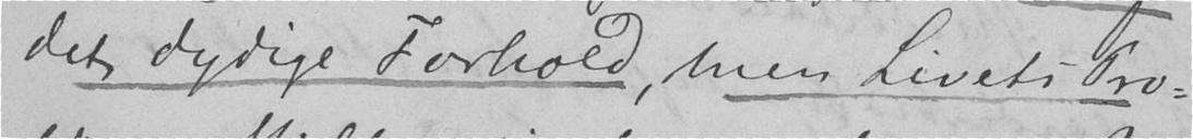det dydige Forhold, men Livets Pro-
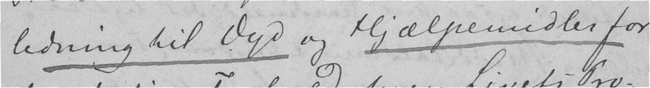ledning til Dyd og Hjælpemidler for
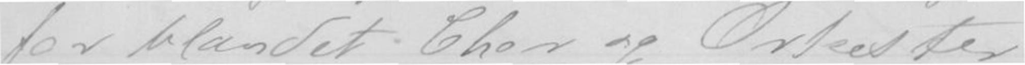for blandet Chor og Orkester
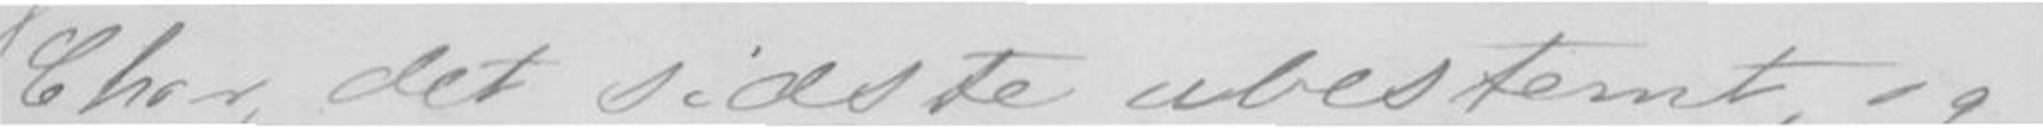Chor, det sidste ubestemt, og
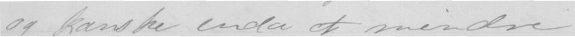og kanske enda et mindre
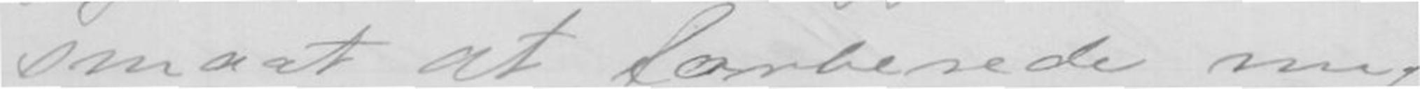smaat at forrberede mig
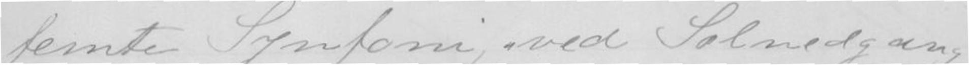femte Symfoni, ved Solnedgang
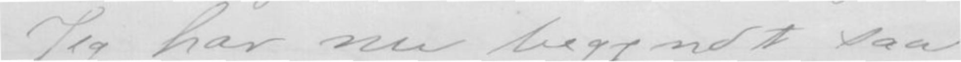Jeg har nu begyndt saa
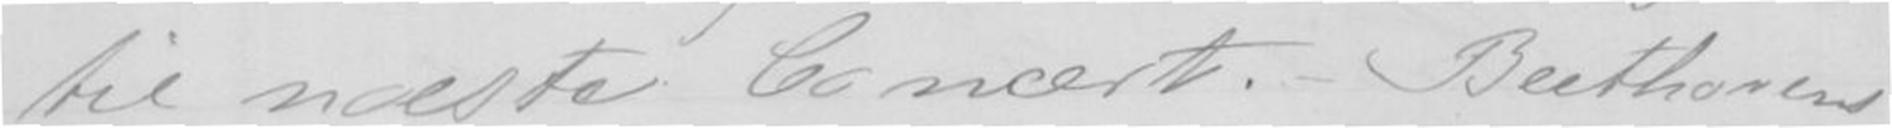til næste Concert. - Beethovens
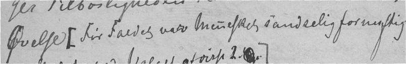Øvelse [Før Faldet var Mennesket sandselig fornuftig
